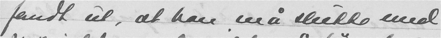fandt ut, at han må slutte med
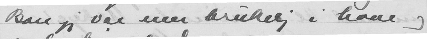Bare jeg var mer brukelig i have og
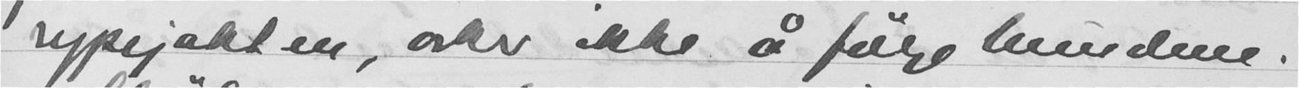rypejakten, orker ikke å følge hundene.
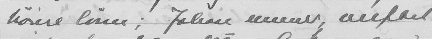høiere lønn; Johan mener, verftet
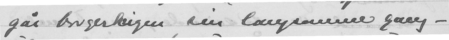går borgerkrigen sin langsomme gang -
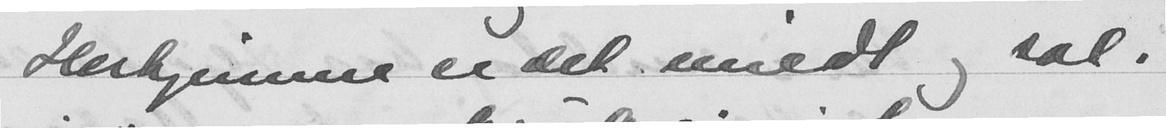Herhjemme er det mildt og sol.
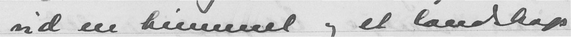vid en himmel og et landskap
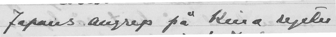Japans angrep på Kina ryster
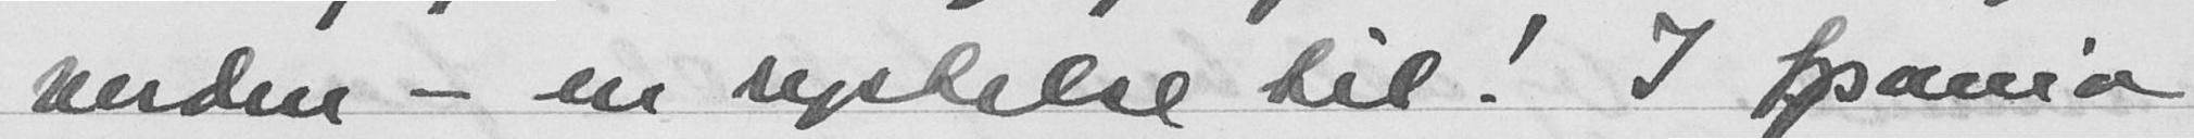verden - en rystelse til! I Spania
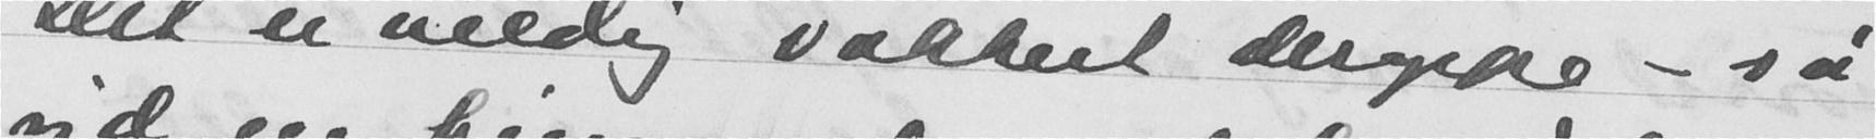Det er veldig vakkert deroppe - så
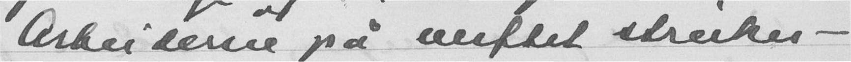Arbeiderne pa verftet streiker -
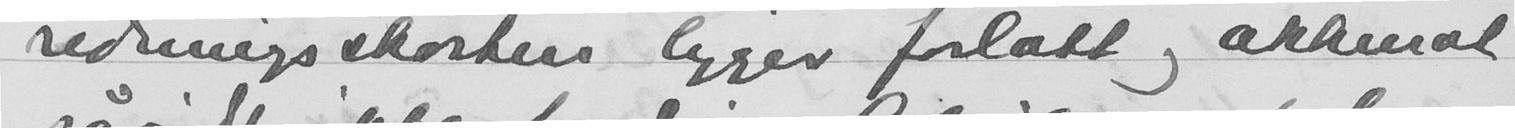redningsskøiten ligger forlatt og akkurat
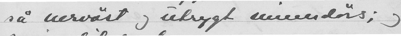så nervøst og utrygt innendørs; og
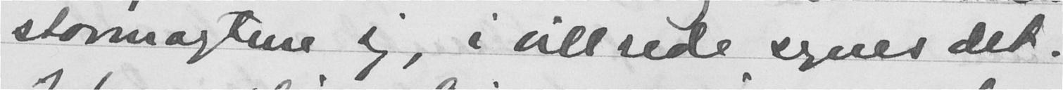stormagtene sig, i villrede synes det.
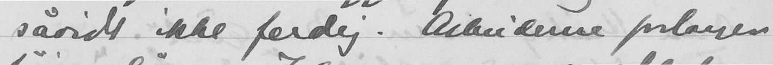såvidt ikke ferdig. Arbeiderne forlanger
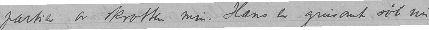partier av skrotten min.  Hans er grusomt søt nu
